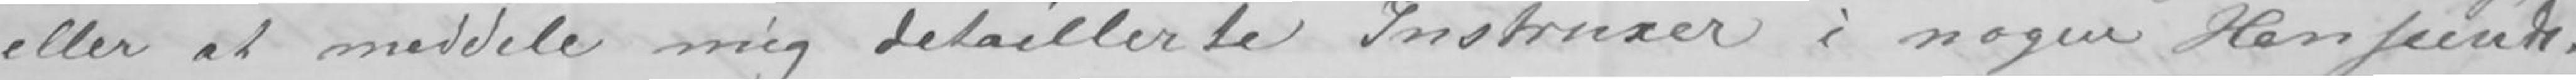eller at meddele mig detaillerte Instruxer i nogen Henseende.
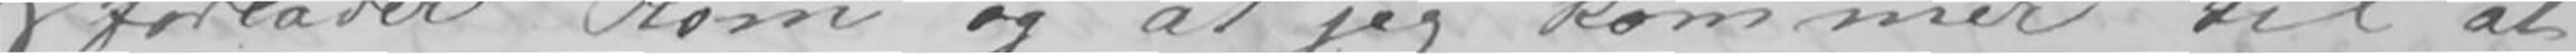forlader Rom og at jeg kommer til at
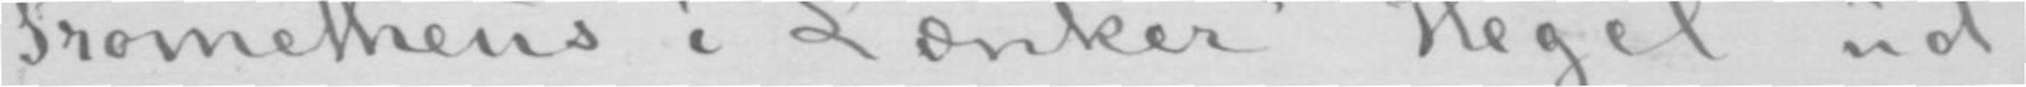«Prometheus i Lænker» Hegel ud-
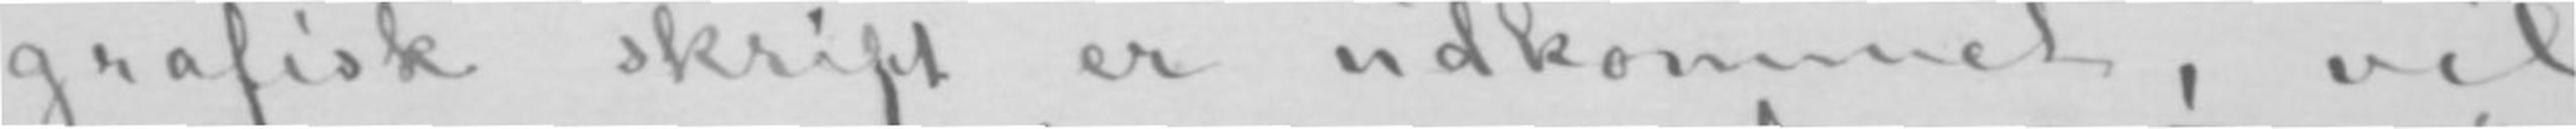grafisk skrift er udkommet, vil
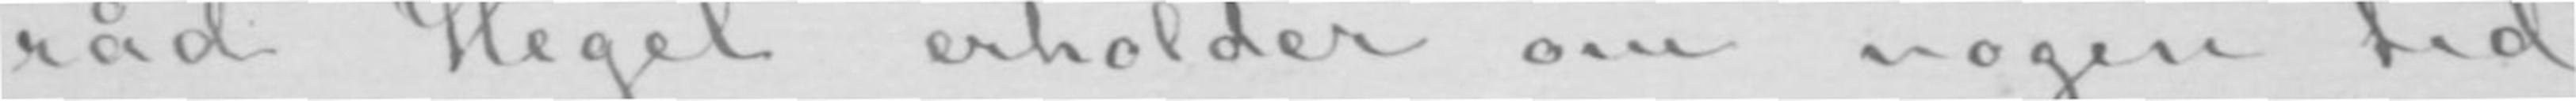råd Hegel erholder om nogen tid
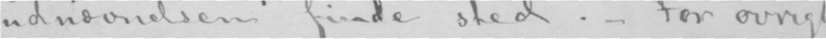udnævnelsen finde sted.- For øvrigt
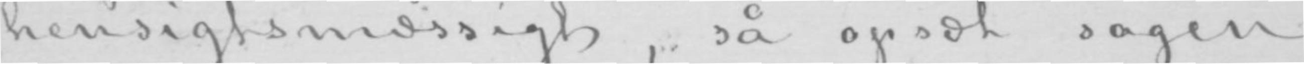hensigtsmæssigt, så opsæt sagen
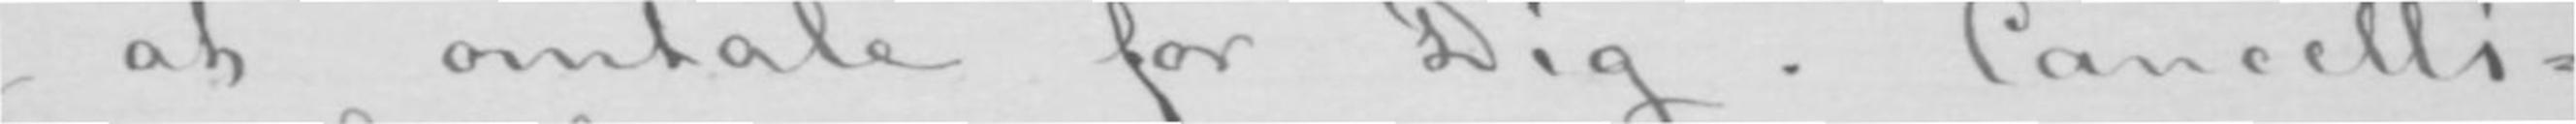jeg at omtale for Dig. Cancelli-
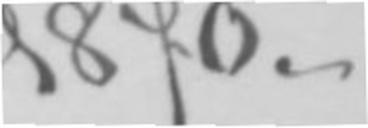1870.
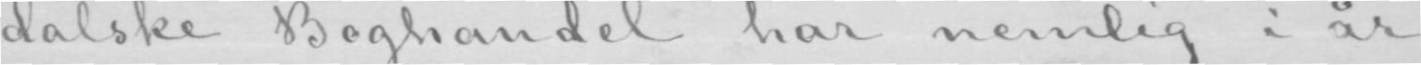dalske Boghandel har nemlig i år
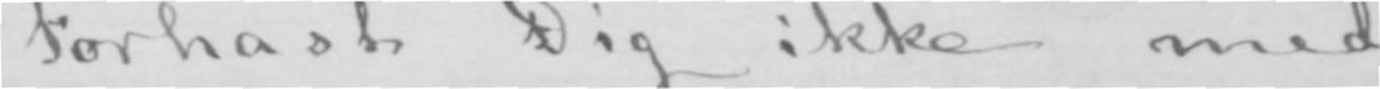Forhast Dig ikke med
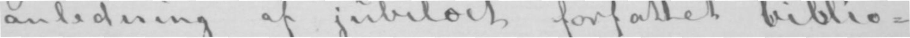anledning af jubilæet forfattet biblio-
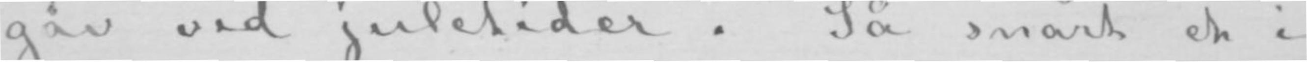gav ved juletider. Så snart et i
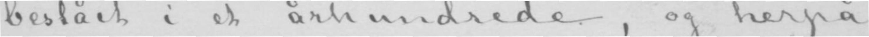bestået i et århundrede, og herpå
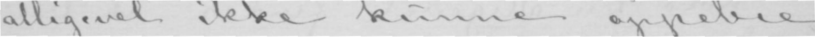alligevel ikke kunne oppebie
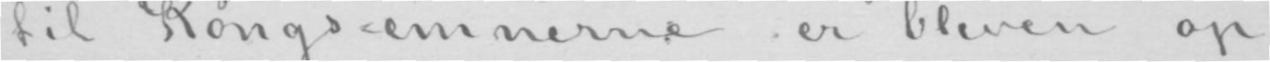til Kongs-emnerne er bleven op-
